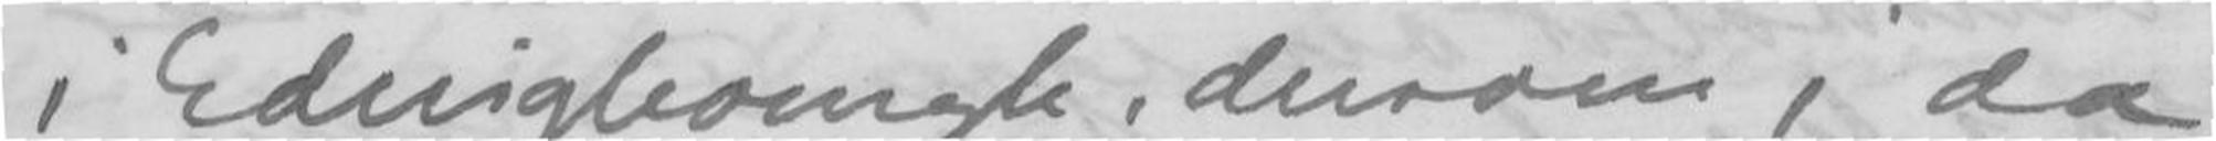i Edingbourgh, derom j da
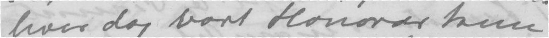hver dag vort Honorar kun
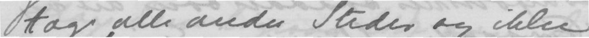alle andre Steder og ikke
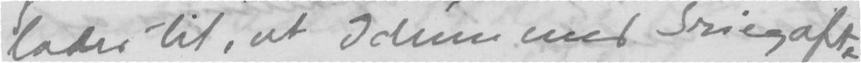lader til, at Ideen med Grieg afte-
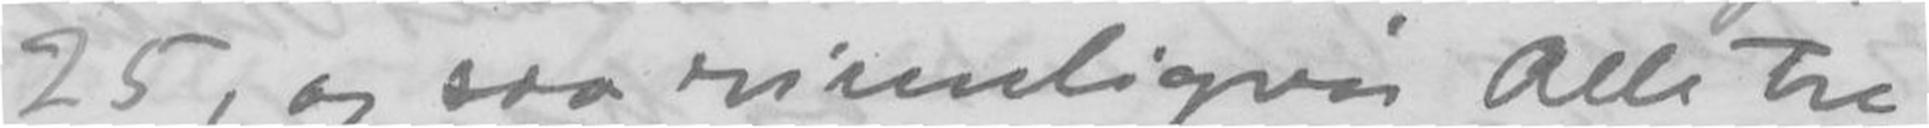25, og saa rimeligvis Alle tre
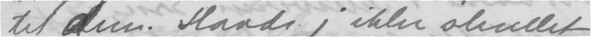til dem. Havde j ikke skullet
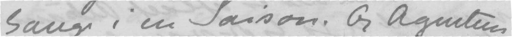Sange i en Saison. Og Agentens
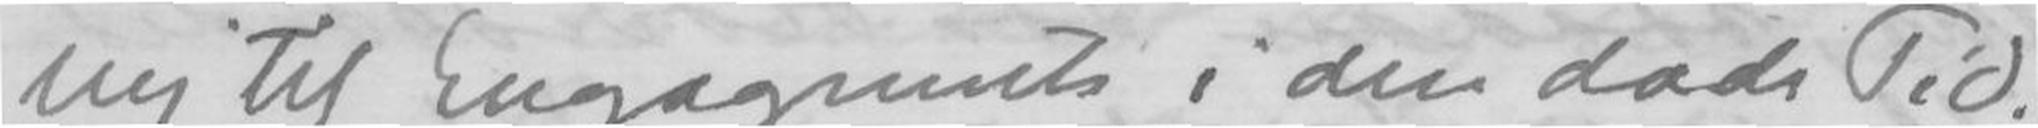nej til Engagements i den døde Tid.
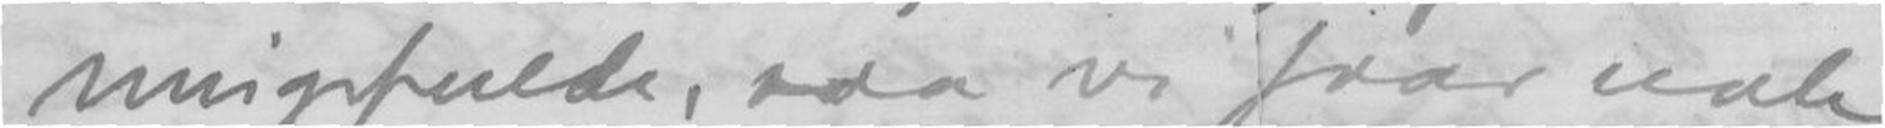ningsfulde, saa vi faar nok
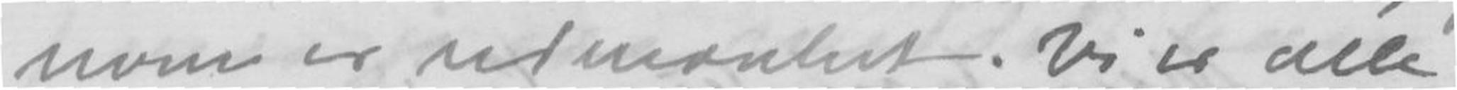nern er udmaerket. Vi er alle
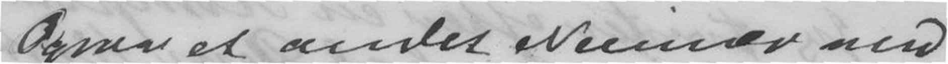Ogsaa et andet Numer med
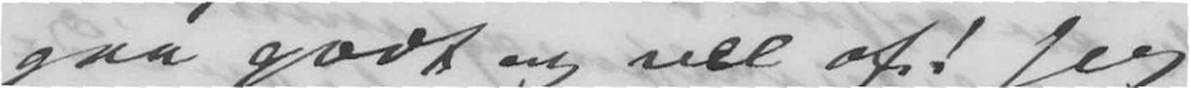gaa godt og vel af! Jeg
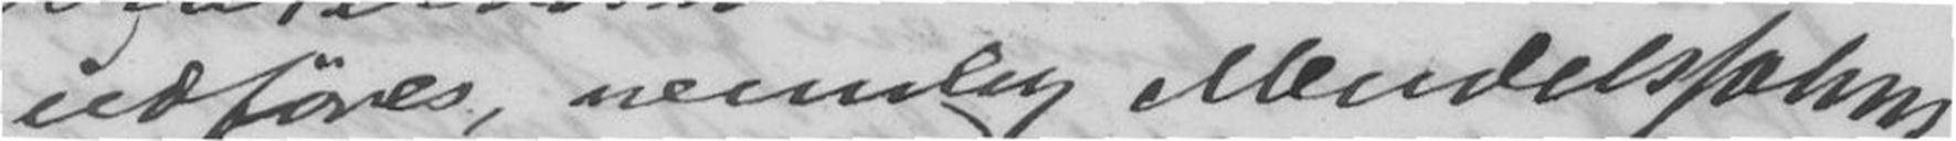udføres, nemlig Mendelsohns
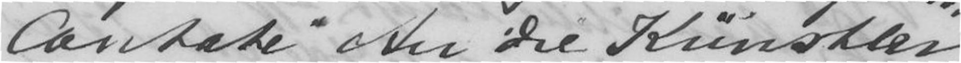Cantate "Au die Künstler"
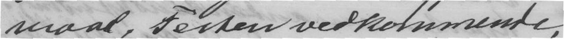maal, Festen vedkommende,
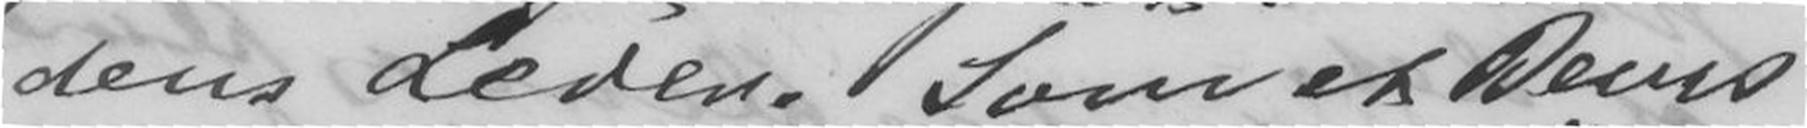dens Leder. Som et Bevis
